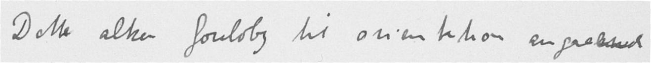Dette altsaa foreløbig til orientation angaaende
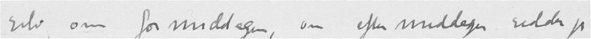selv om formiddagen, om eftermiddagen sidder jeg
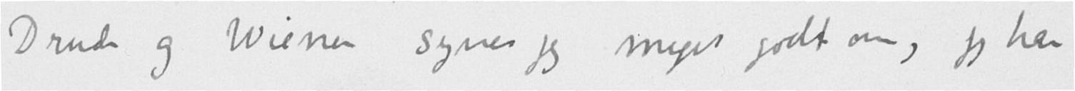Drude og Wiener synes jeg meget godt om, jeg har
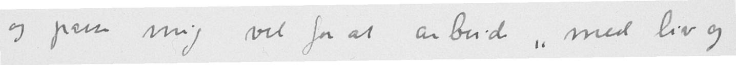og passe mig vel for at arbeide "med liv og
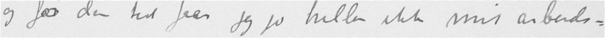og før den tid faar jeg jo heller ikke mit arbeids-
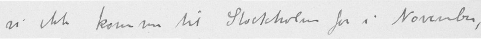vi ikke komme til Stockholm før i November,
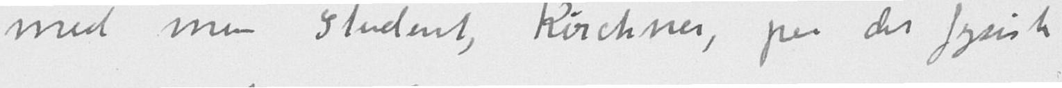med min student, Kirchner, paa det fysiske
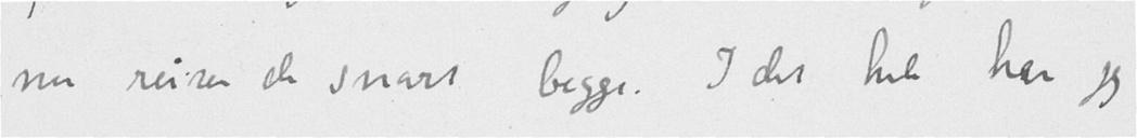nu reiser de snart begge. I det hele har jeg
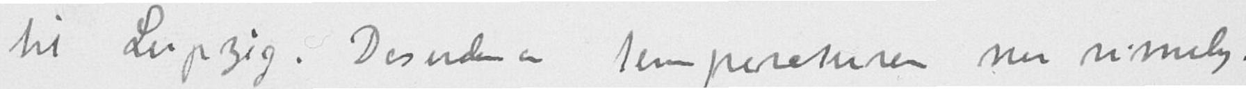til Leipzig. Desuden er temperaturen nu rimelig.
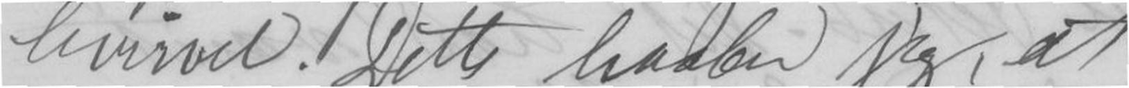hvirvel. Dette haaber jeg, at
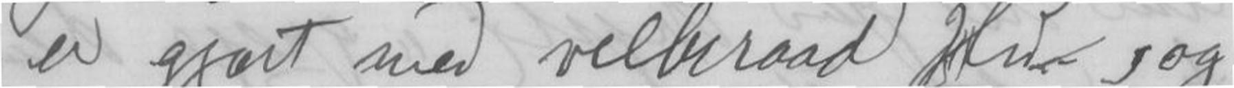er gjort med velberaad Hu, og
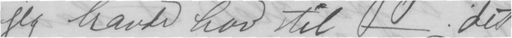jeg havde Lov til - det
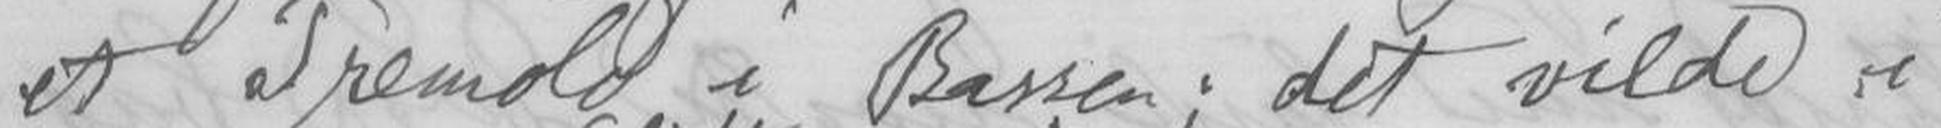et Tremolo i Bassen; det vilde i
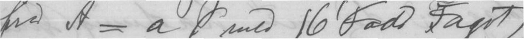fra A=a (med 16 Fods Fagot)
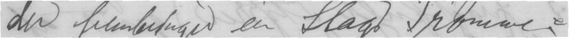der frembringer ein Slags Tromme-
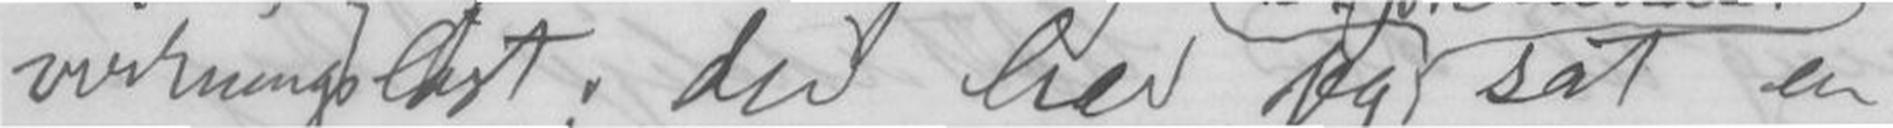virkningsløst; der har jeg sat en
